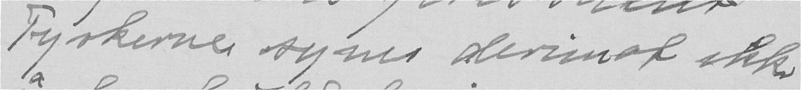Tyskerne synes derimot ikke
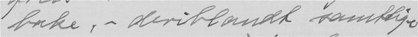bake, - deriblandt samtlige
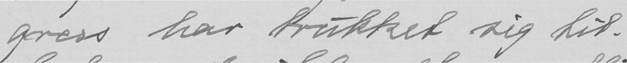gress har trukket sig til-
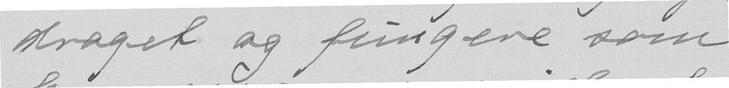draget og fungere som
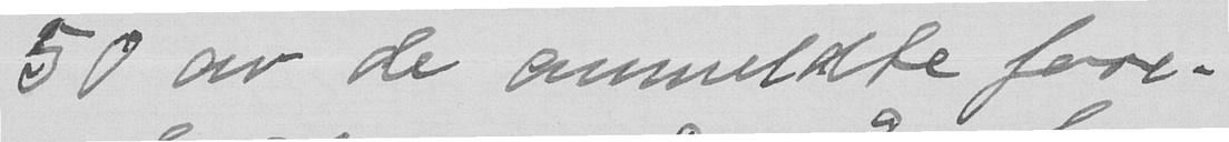50 av de ammeldte fore-
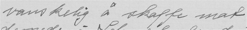vanskelig å skaffe mat
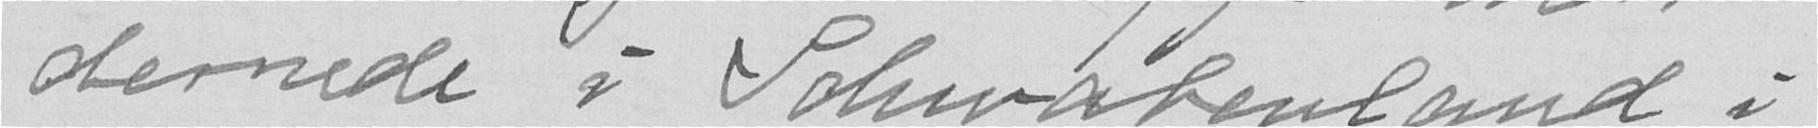dernede i Schwabenland i
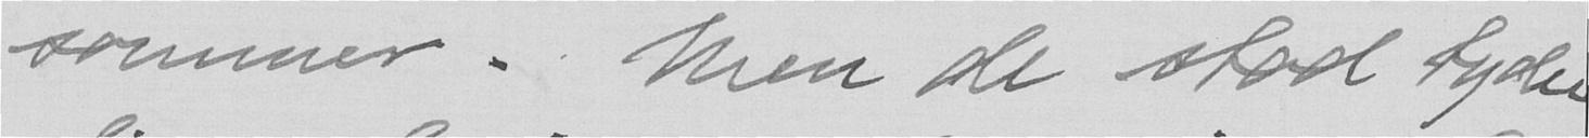sommer. Men de stod tyde-
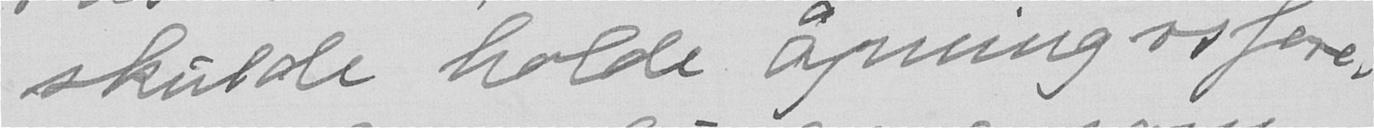skulde holde åpningsfore-
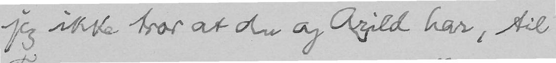jeg ikke tror at du og Arild har, til
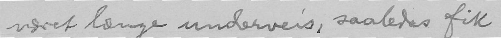været længe underveis, saaledes fik
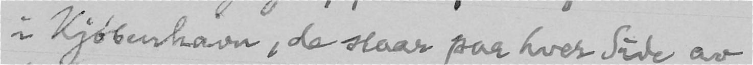i Kjøbenhavn, de staar paa hver Side av
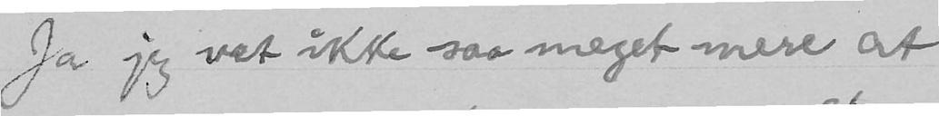Ja jeg vet ikke saa meget mere at
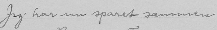Jeg har nu sparet sammen
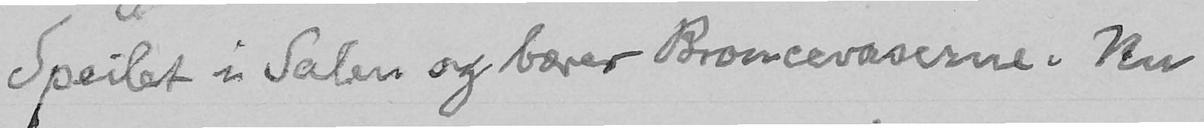Speilet i Salen og bærer Broncevaserne. Nu
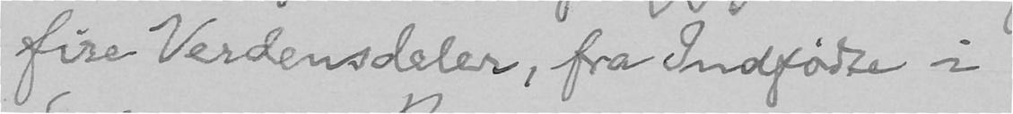fire Verdensdeler, fra Indfødte i
